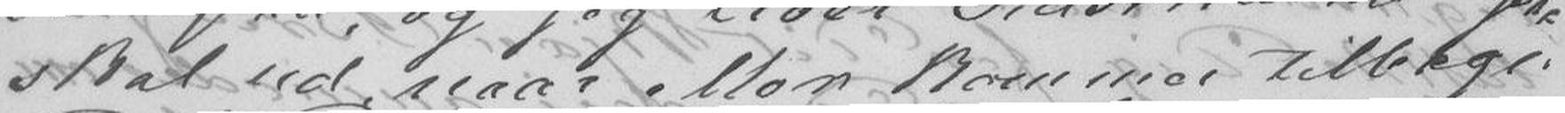skal ud naar Mor kommer tilbage.
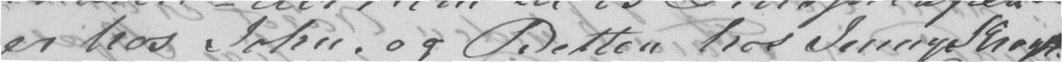er hos John, og Betten hos Jenny Krogh.
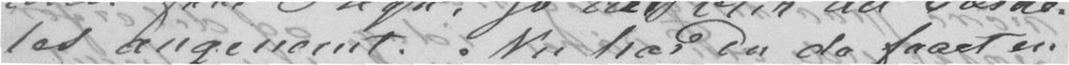les angenemt. Nu har Du da faaet en
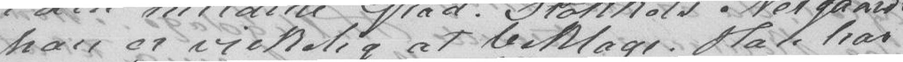han er virkelig at beklage. Han har
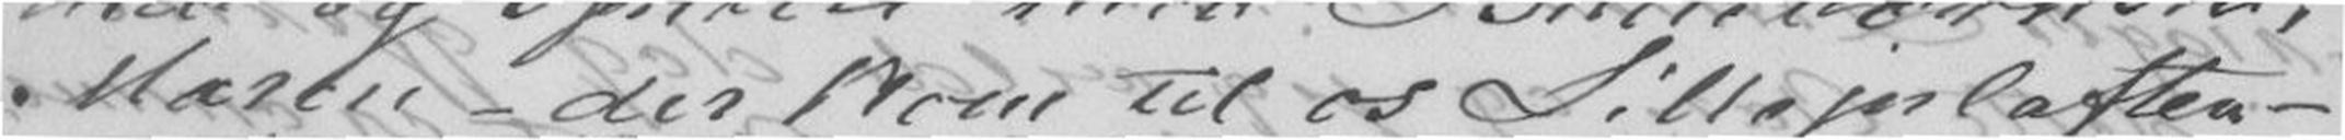Maren - der kom til os Lillejulaften -
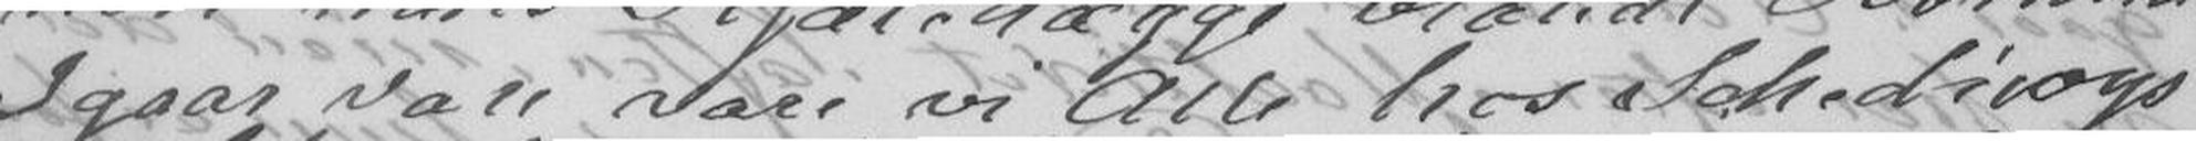Igaar vare vare vi Alle hos Schediwys
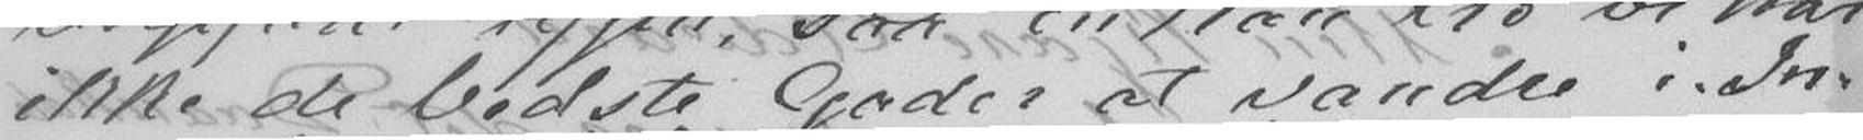ikke de bedste Goder at vandre i. In-
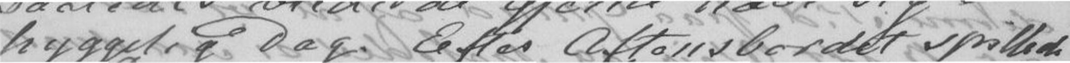hyggelig Dag. Efter Aftensbordet spillede
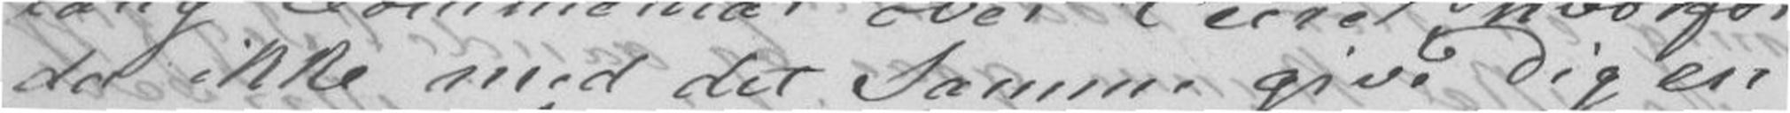da ikke med det Samme give Dig en
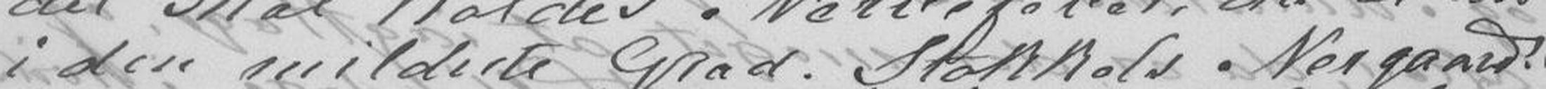i den mildeste Grad. Stakkels Nergaards
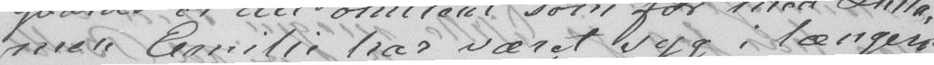men Emilie har været syg i længere
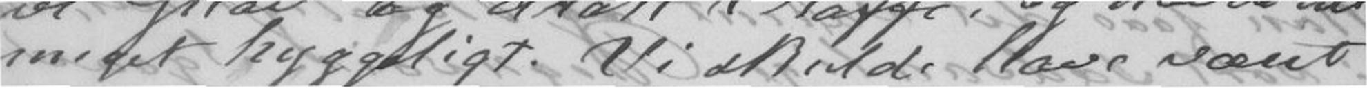meget hyggeligt. Vi skulde have været
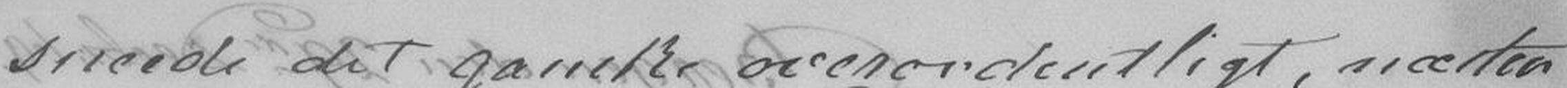sneede det ganske overordentlig, næsten
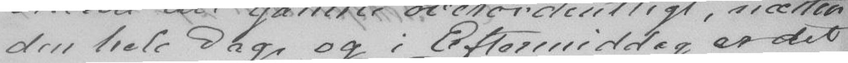den hele Dag og i Eftermiddag er det
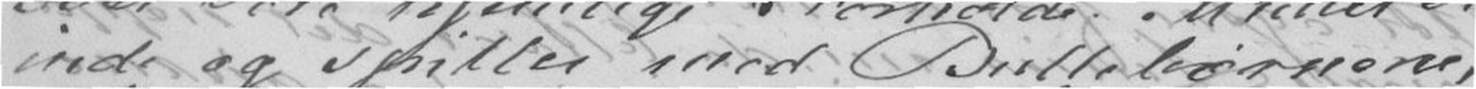inde og spiller med Bullebørnene,
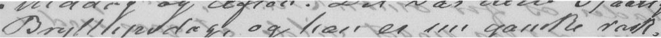Bryllupsdag, og han er nu ganske rask
